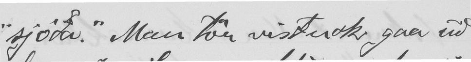"sjóða". Man tør vistnok gaa ud
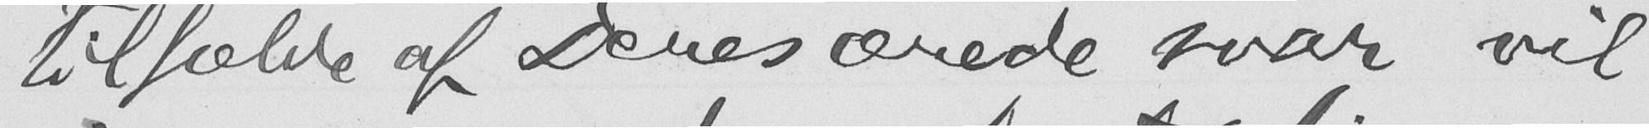tilfælde af Deres ærede svar vil
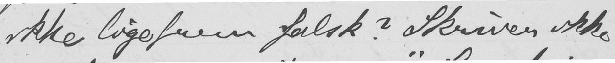ikke ligefrem falsk? Skriver ikke
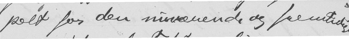pelt for den nuværende og fremtidige
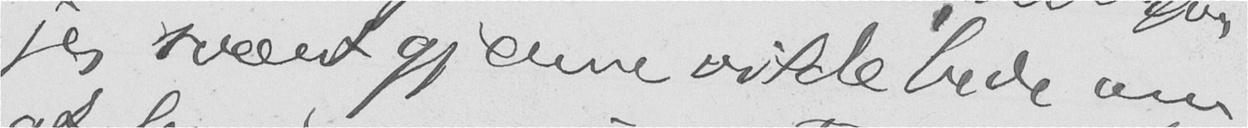jeg svært gjerne vilde bede om
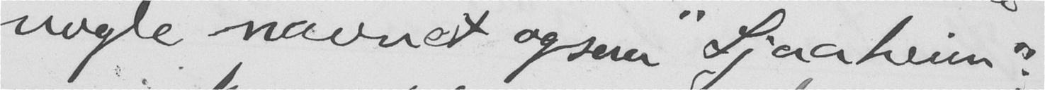nogle navnet ogsaa "Sjaaheim";
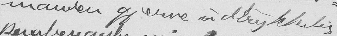manden gjerne udtrykkelig
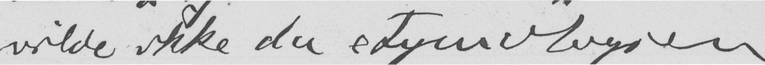vilde ikke da etymologien
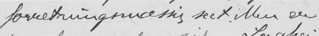forretningsmæssig seet. Men er
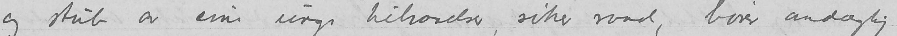og stub av sine unge tilværelser, søker raad, hører andægtig
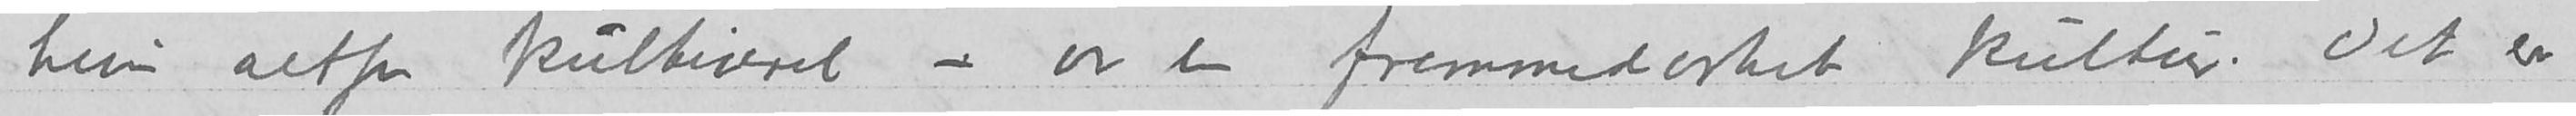hun altfor kultivert – av en fremmedartet kultur. Det er
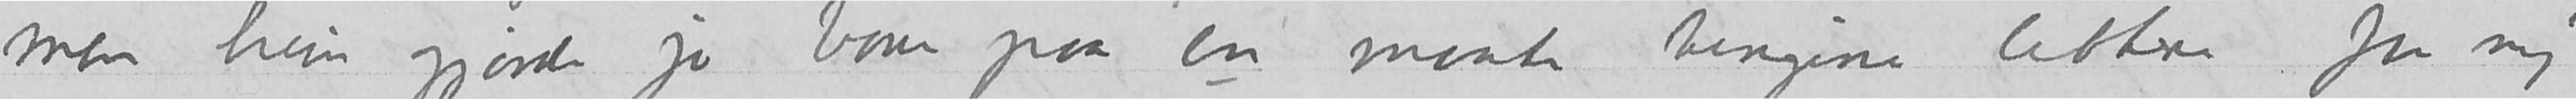men hun gjorde jo bare paa en maate tingene lettere for mig
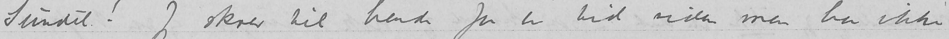Sundil! Jeg skrev til hende for en tid siden men har ikke
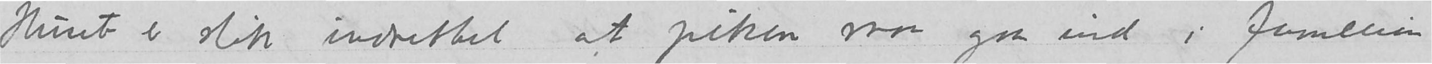Huset er slik indrettet at piken maa gaa ind i familien
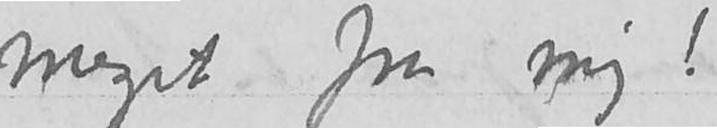saa meget fra mig!
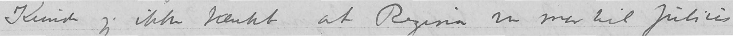Kunde jeg ikke tænkt at Regine var mor til Julius
